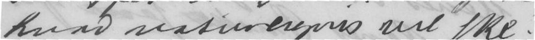hvad naturligvis vil ske!
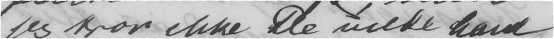jeg tror ikke De vilde have
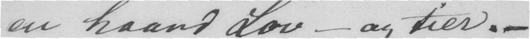en haard Lov - og tier. -
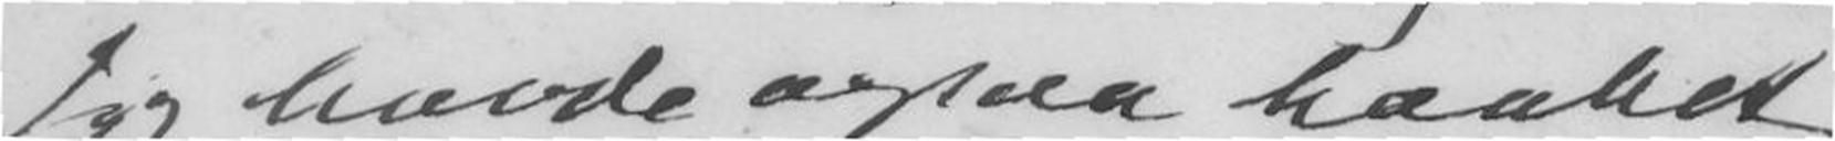Jeg havde ogsaa haabet
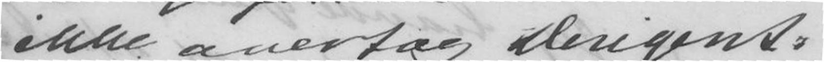ikke overtog Dirigent-
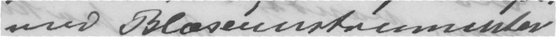med Blæseinstrumenter
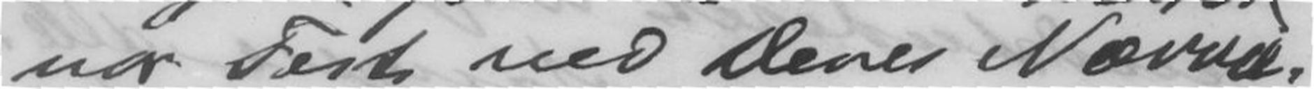vor Fest ved Deres Nærvæ-
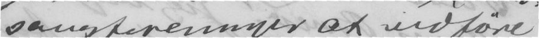sangforeninger at udføre
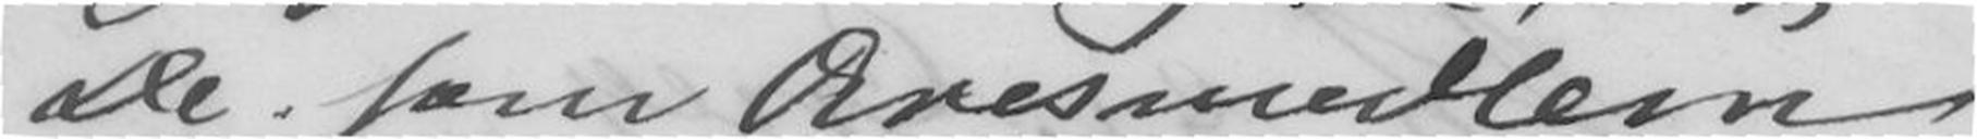De som Æresmedlem
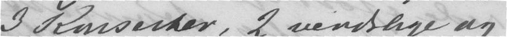3 Konserter, 2 verdslige og
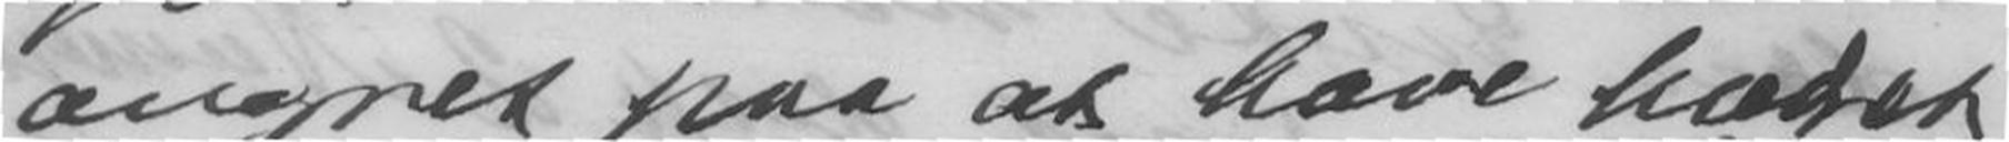angret paa at have hædret
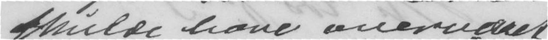skulde have overværet
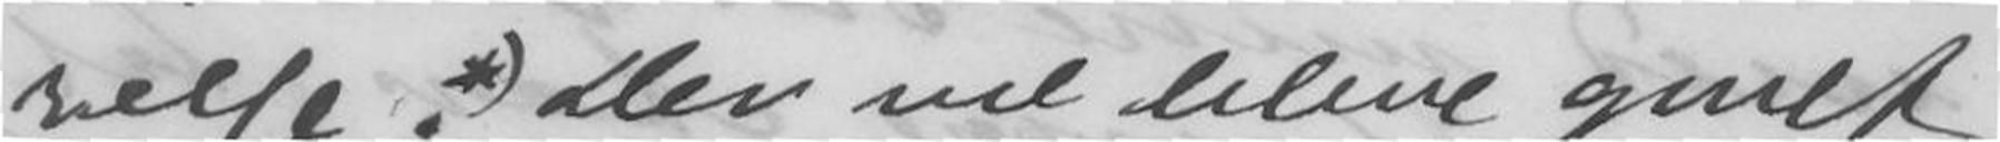relse. *)Der vil blive givet
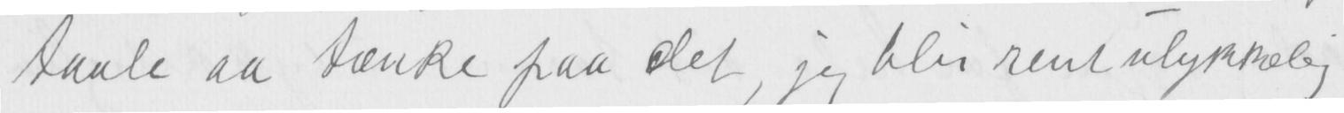taale aa tænke paa det, jeg blir rent ulykkelig.
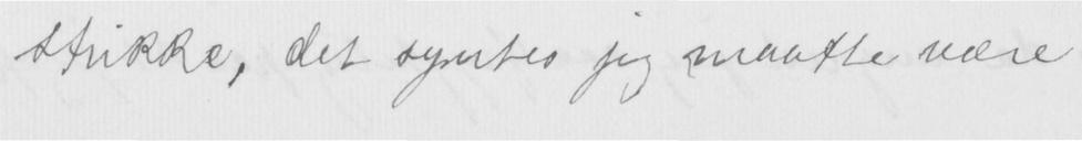strikke, det syntes jeg maatte være
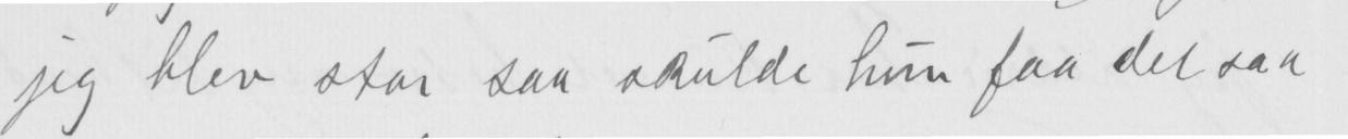jeg blev stor saa skulde hun faa det saa
